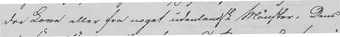dre Love eller fra noget udenlandsk Mønster. Dens
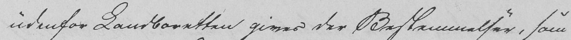udenfor Landboretten gives der Bestemmelser, som
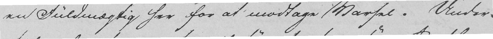en Fuldmægtig her for at modtage Varsel. Under-
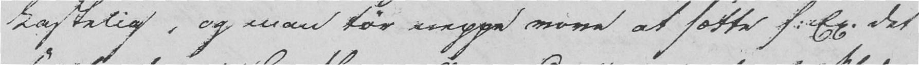kastelig, og man tør neppe vove at sætte f. Ex. det
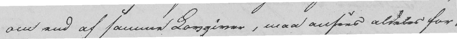om end af samme Lovgiver, maa ansees aldeles for-
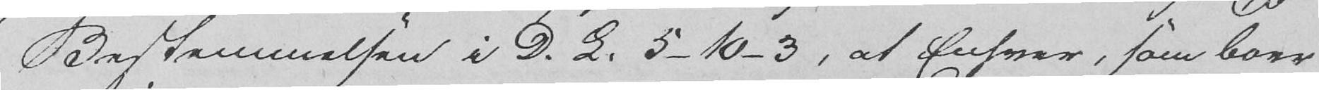Bestemmelsen i D. L. 5-10-3, at Enhver, som boer
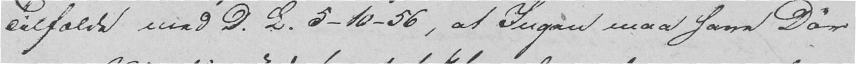Tilfælde med D. L. 5-10-56, at Ingen maa have Dør
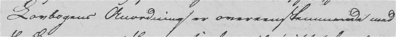Lovbogens Anordning er overeenstemmende med
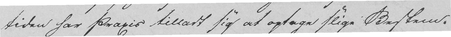tiden har Praxis tilladt sig at optage slige Bestem-
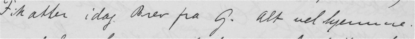Fik atter idag Brev fra G. Alt vel hjemme.
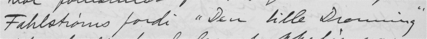Fahlstrøms fordi "Den lille Dronning"
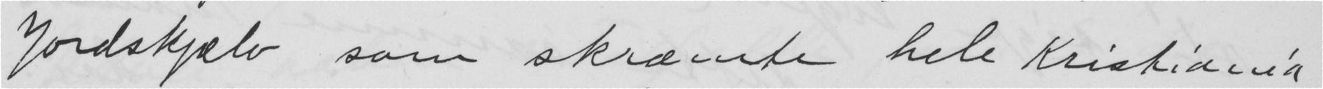Jordskjælv som skræmte hele Kristiania
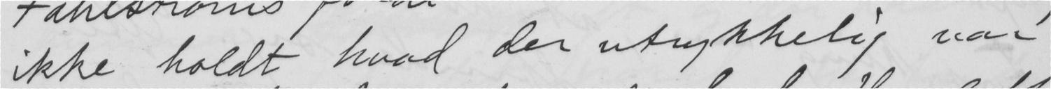ikke holdt hvad der utrykkelig var
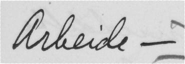Arbeide -
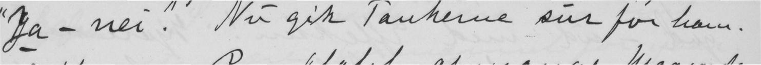"Ja - nei". Nu gik Tankerne sur for ham.
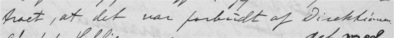troet, at det var forbudt af Direktøreren.
